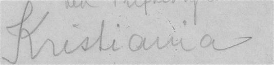Kristiania
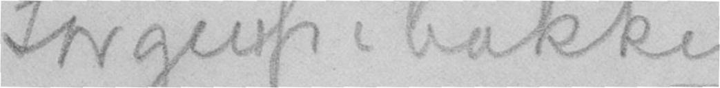Sorgenfribakken
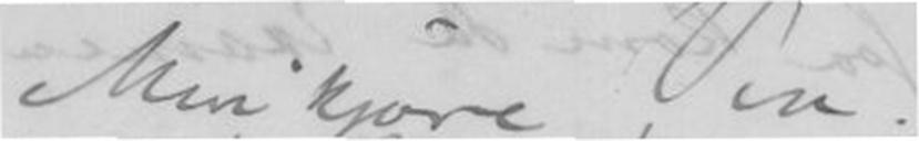Min kjære Ven.
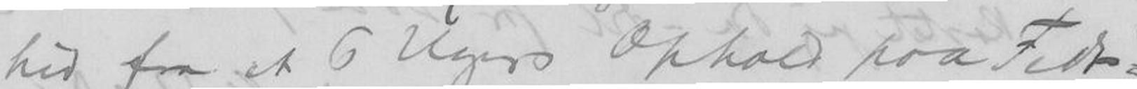hid fra et 6 Ugers Ophold paa Fedr-
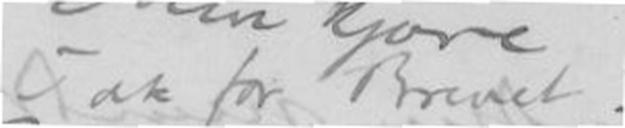Tak for Brevet!
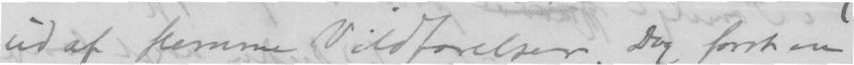ud af slemme Vildfarelsen. Dog først en
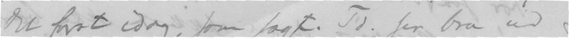det først idag, som sagt. Td. ser bra ud
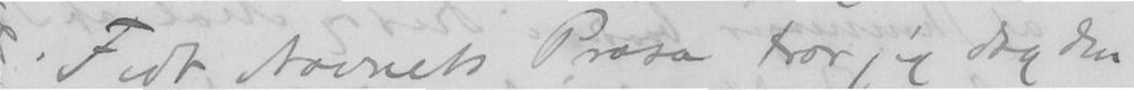Fedr Navnet Prosa tror jeg dog den
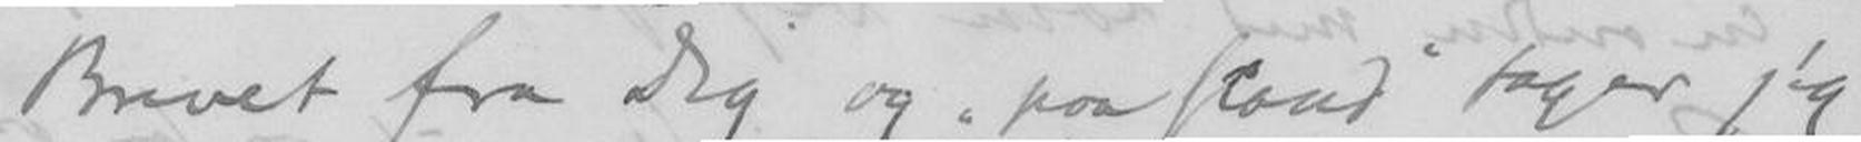Brevet fra dig og paastand tager jeg
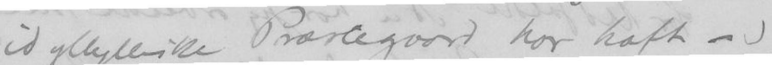idyllylliske Præstegaard har haft -
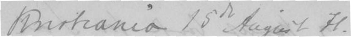Kristiania 15de August 71.
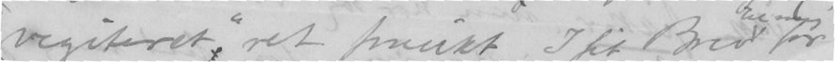vegiteret" ret smukt I sit Brev for
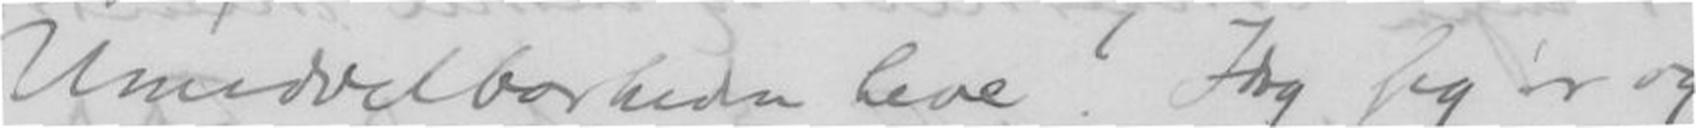Umiddelbarheden leve! Idag siger og
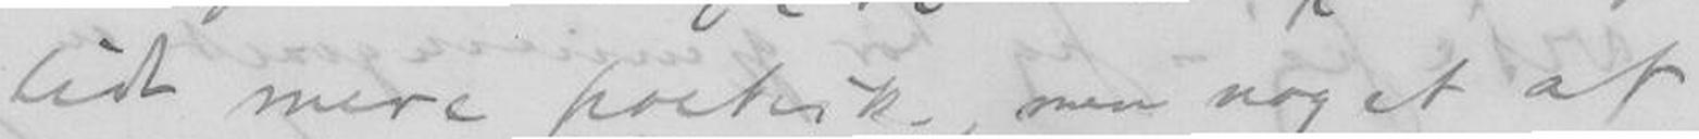lidt mere poetisk, men noget af
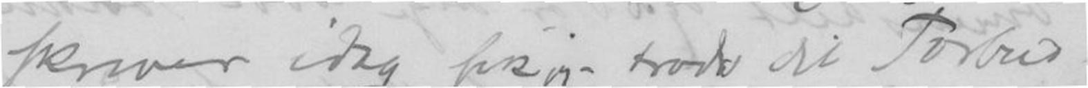skriver idag fik jeg trods dit Forbud.
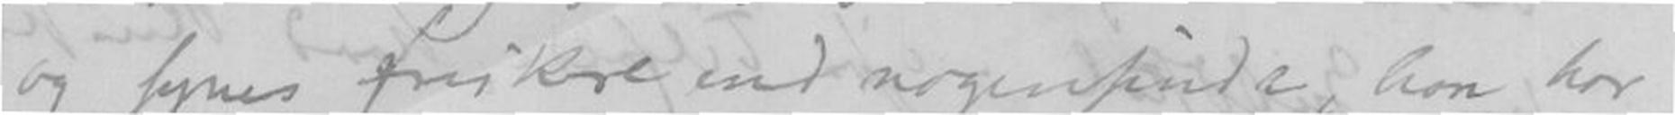og synes friskere end nogensinde, han har
